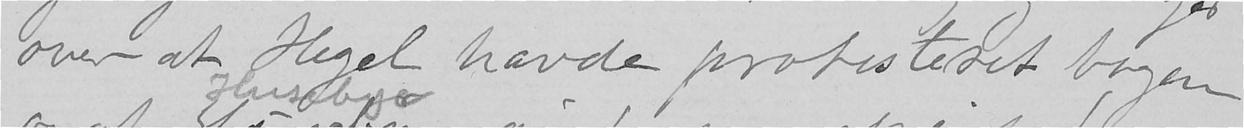over at Hegel havde protesteret bogen
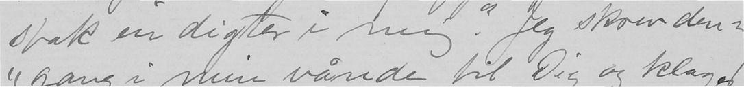"stak en digter i mig". Jeg skrev den-
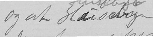og at Husebye
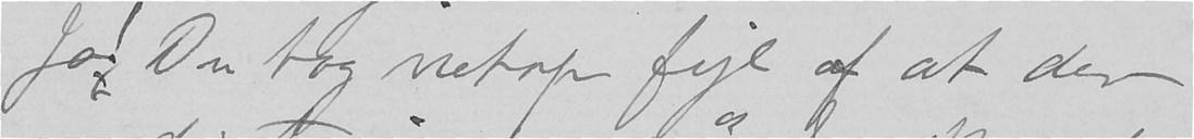Jo! Du tog netop fejl af at der
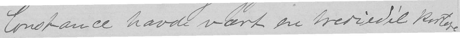Constance havde vært en trediedel kortere
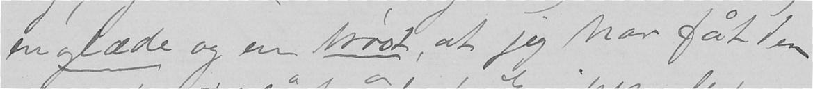en glæde og en trøst, at jeg har fåt den
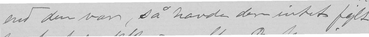end den var, så havde der intet fejlt
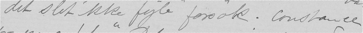det slet ikke "fejle" forsøk. Constance
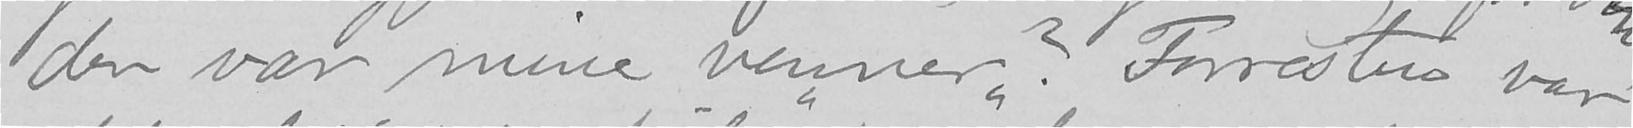der var mine venner? Forresten var
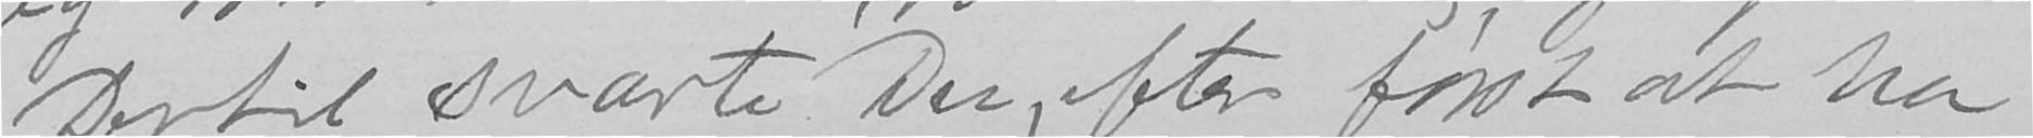Dertil svarte Du, efter først at ha
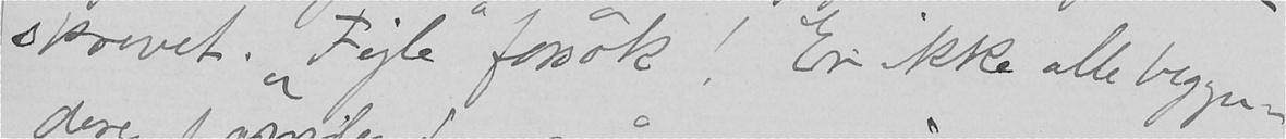skrevet. "Fejle" forsøk! Er ikke alle begyn-
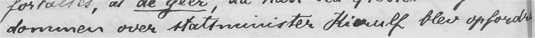dommen over statsminister Kierulf blev opford
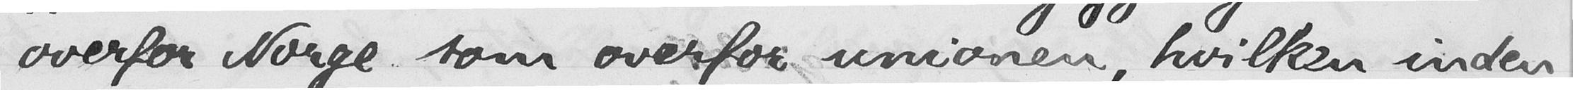overfor Norge som overfor unionen, hvilken inden
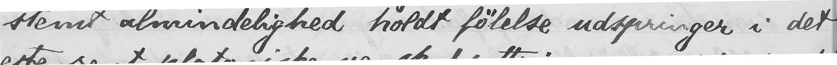stemt almindelighed holdt følelse udspringer i det
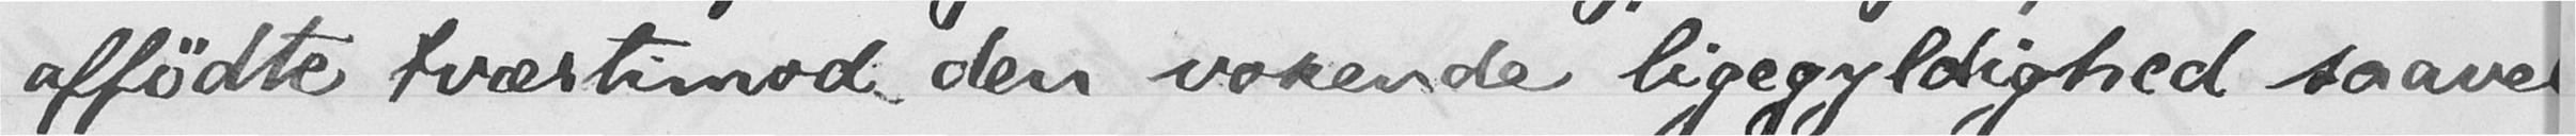affødte tværtimod den voxende ligegyldighed saavelsom
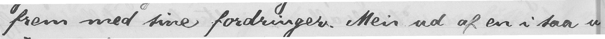frem med sine fordringer. Men ud af en i saa
